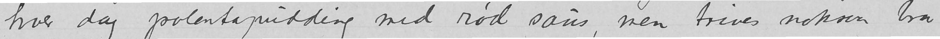hver dag polentapudding med rød saus, men trives noksaa bra
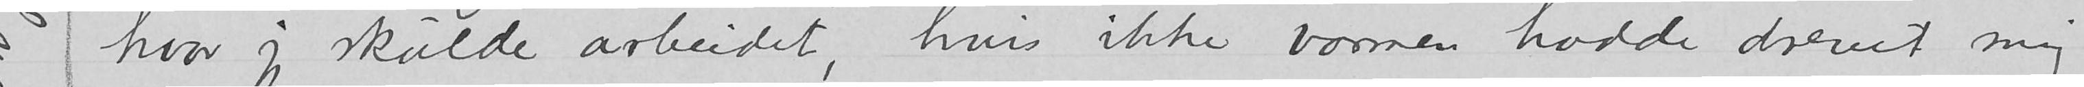hvor jeg skulde arbeidet, hvis ikke varmen hadde drevet mig
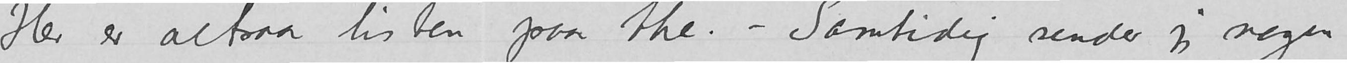Her er altsaa listen paa the. – Samtidig sender jeg nogen
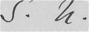S. U.
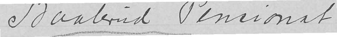Baalerud Pensionat
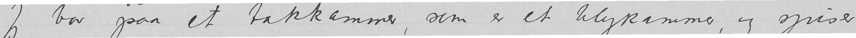Jeg bor paa et takkammer, som er et blykammer, og spiser
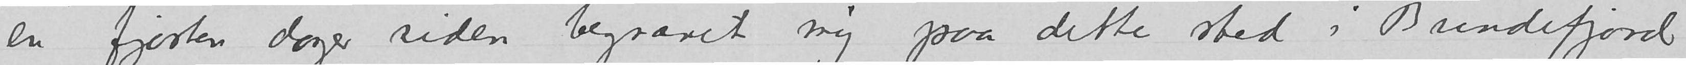en fjorten dager siden begravet mig paa dette sted i Bundefjord,
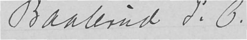Baalerud P. B.
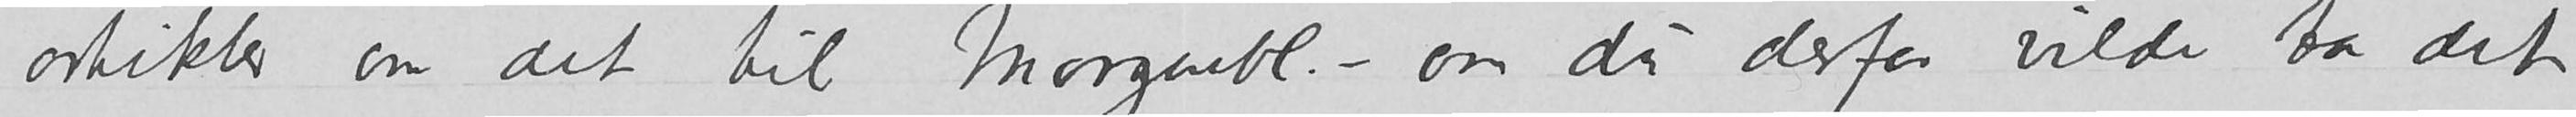artikler om det til Morgenbl. – om du derfor vilde ta det
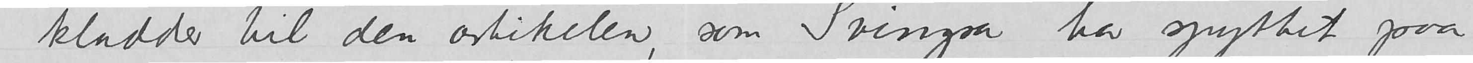kladder til den artikelen, som Svingsen har spyttet paa
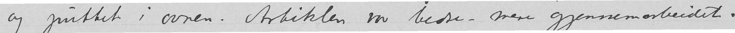og puttet i ovnen. Artikelen var bedre – mere gjennemarbeidet.
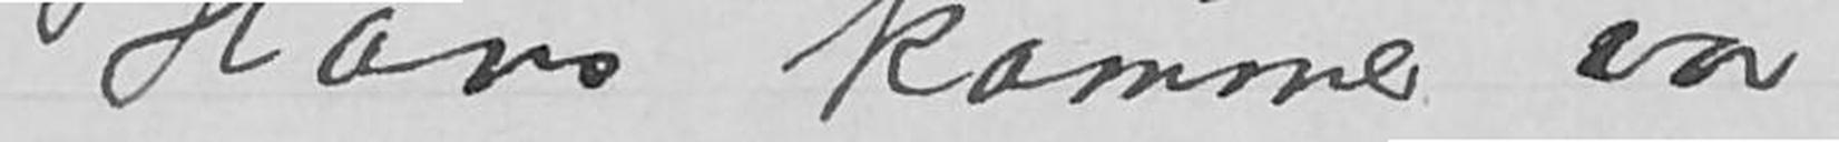Hans kammer var
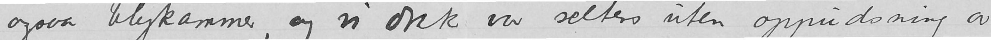ogsaa blykammer, og vi drak vor selters uten oppudsning av
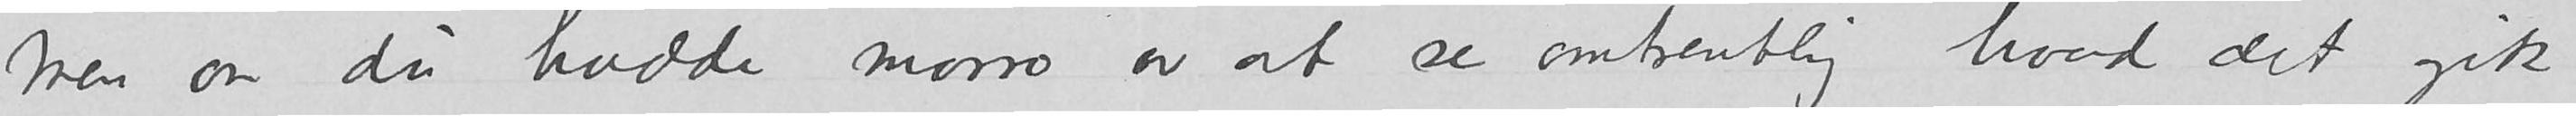Men om du hadde morro av at se omtrentlig hvad det gik
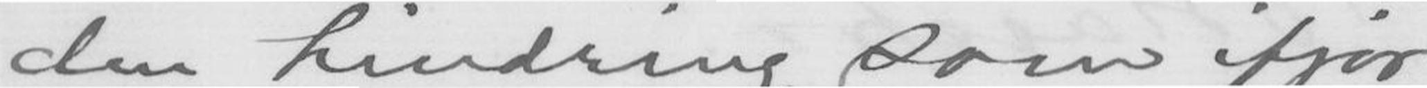den hindring, som ifjor
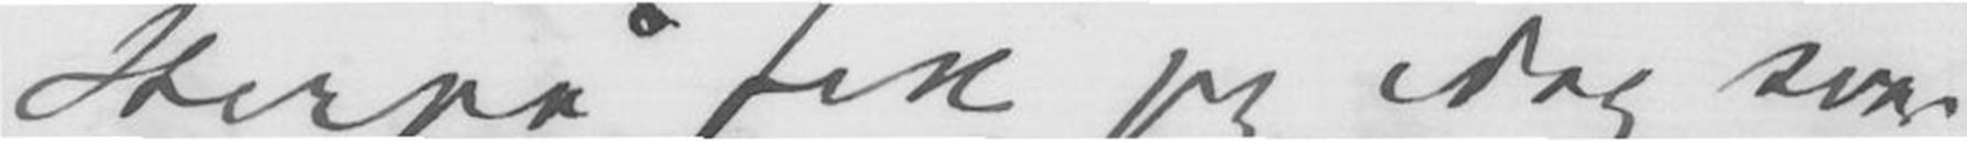Herpå fin jeg idag svar
